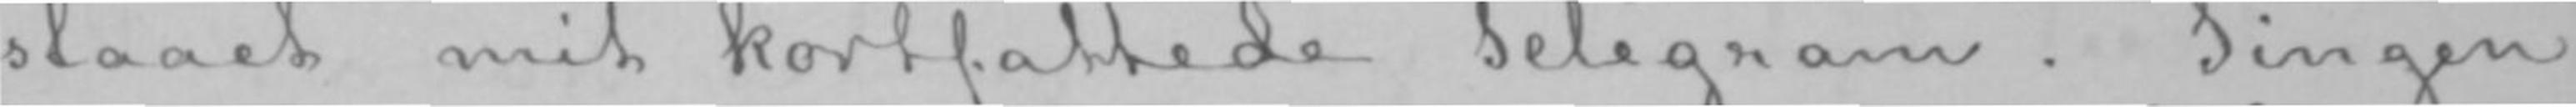staaet mit kortfattede Telegram. Tingen
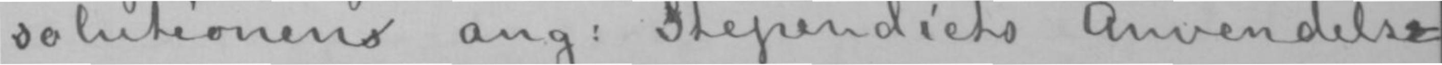solutionens ang: Stipendiets Anvendelse
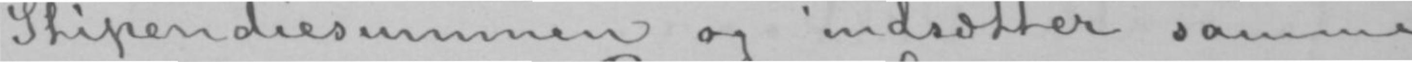Stipendiesummen og indsætter samme
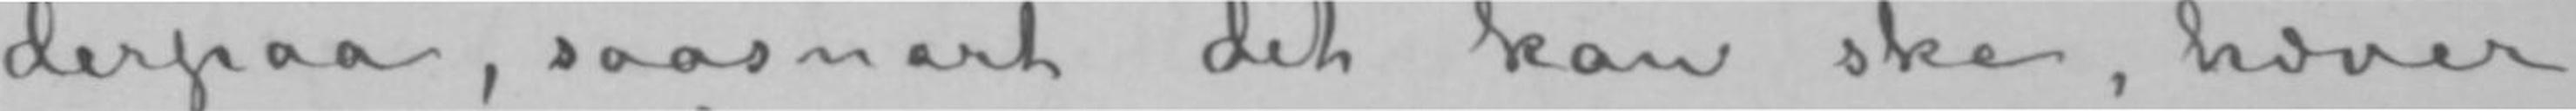derpaa, saasnart det kan ske, hæver
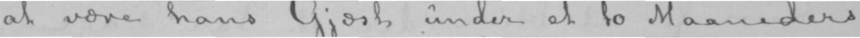at være hans Gjæst under et to Maaneders
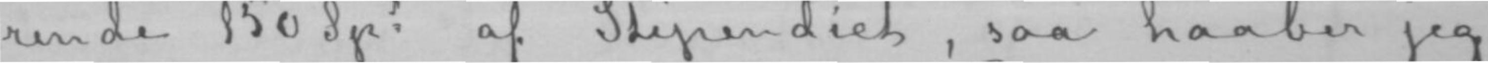rende 150 Spd af Stipendiet, saa haaber jeg
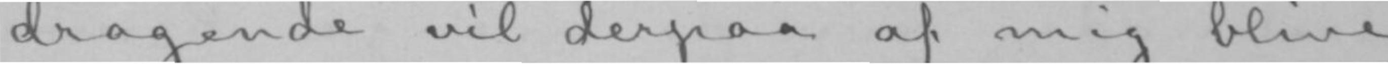dragende vil derpaa af mig blive
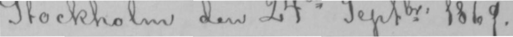Stockholm den 24de Septbr: 1869.-
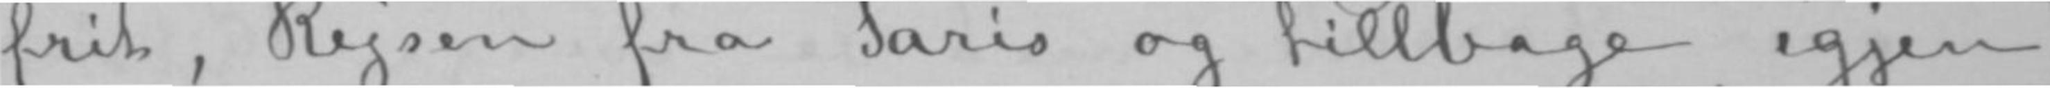frit, Rejsen fra Paris og tillbage igjen
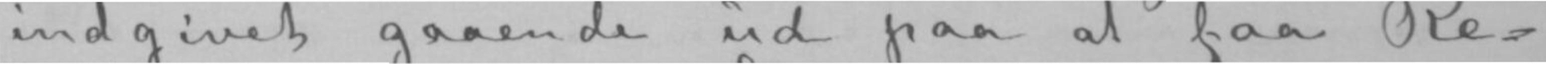indgivet gaaende ud paa at faa Re-
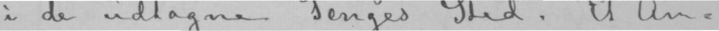i de udtagne Penges Sted. Et An-
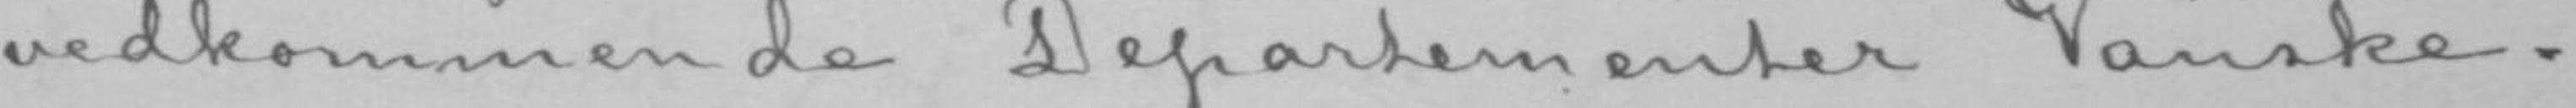vedkommende Departementer Vanske-
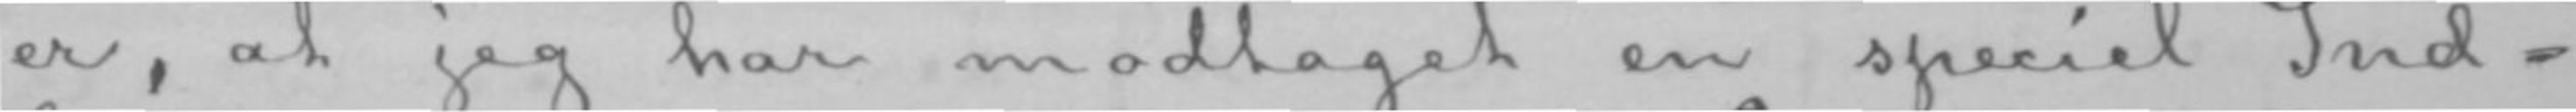er, at jeg har modtaget en speciel Ind-
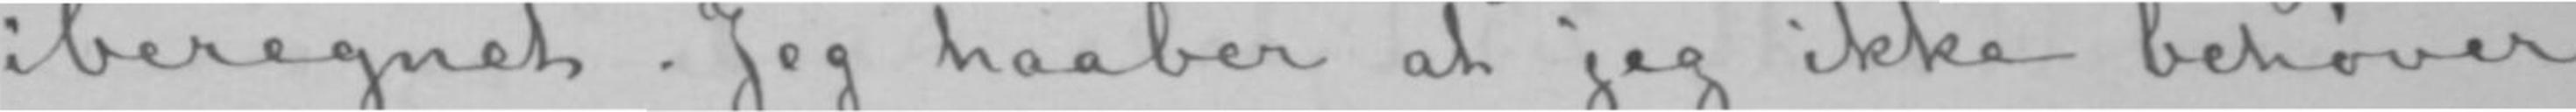iberegnet. Jeg haaber at jeg ikke behøver
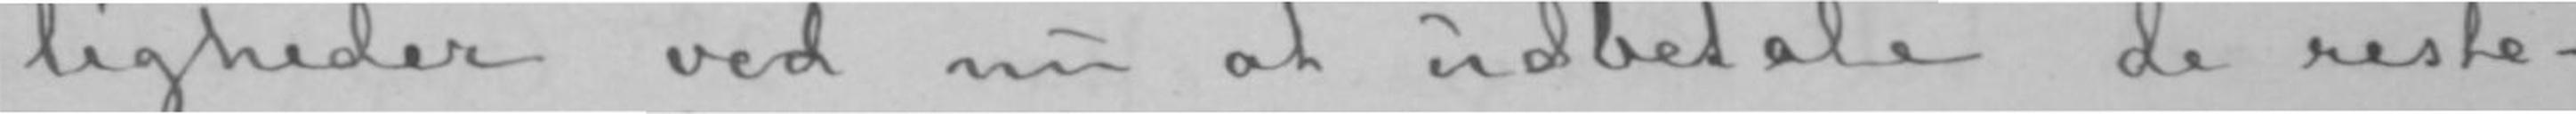ligheder ved nu at udbetale de reste-
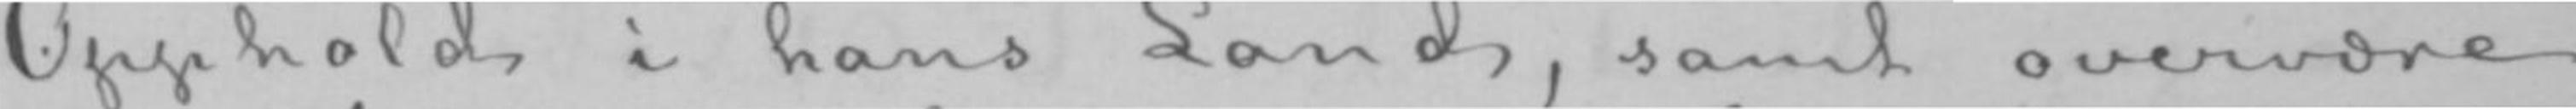Opphold i hans Land, samt overvære
